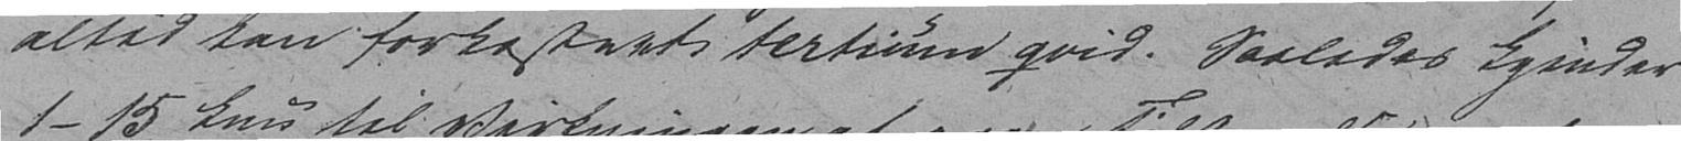altid kan forkastert tertium quid. Saaledes kjender
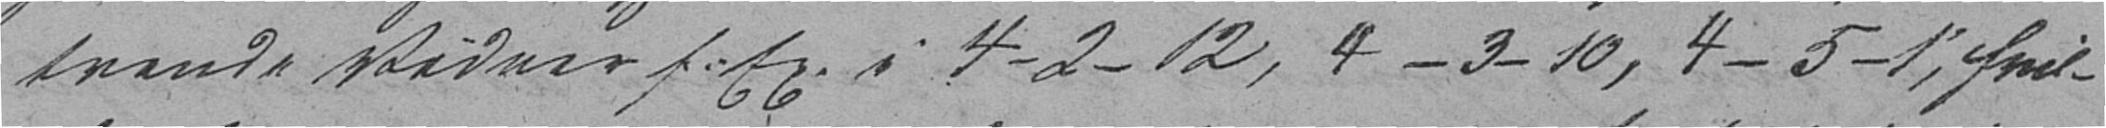tvende Vidner f. Ex. i 4-2-12, 4-3-10, 4-5-1, hvil-
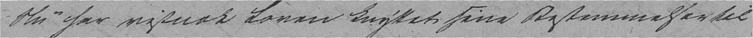Nu har vistnok Loven knyttet sine Bestemmelser til
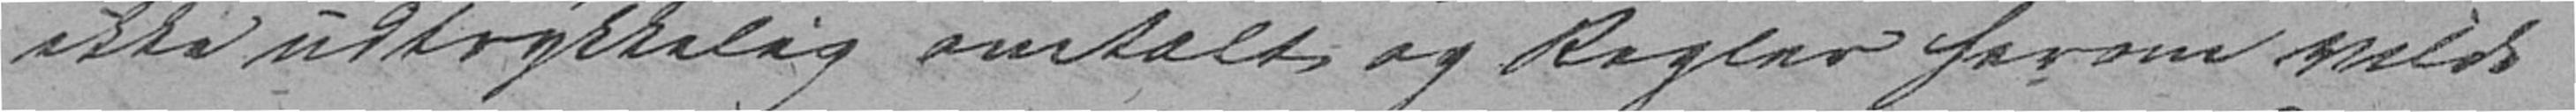ikke udtrykkelig omtalt, og Regler herom vilde
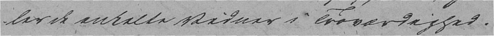ler de enkelte Vidner i Troværdighed.
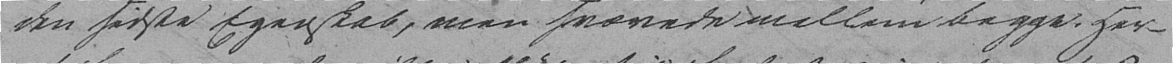den sidste Egenskab, men svarede mellem begge. Her-
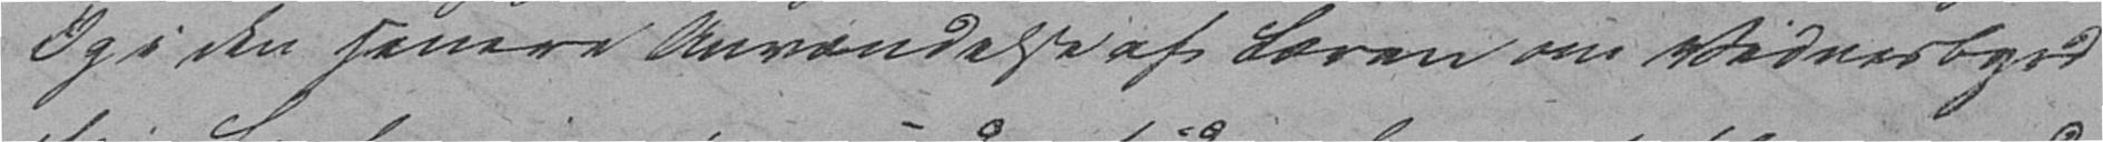Og i den senere Anvendelse af Loven om Vidnesbyrd
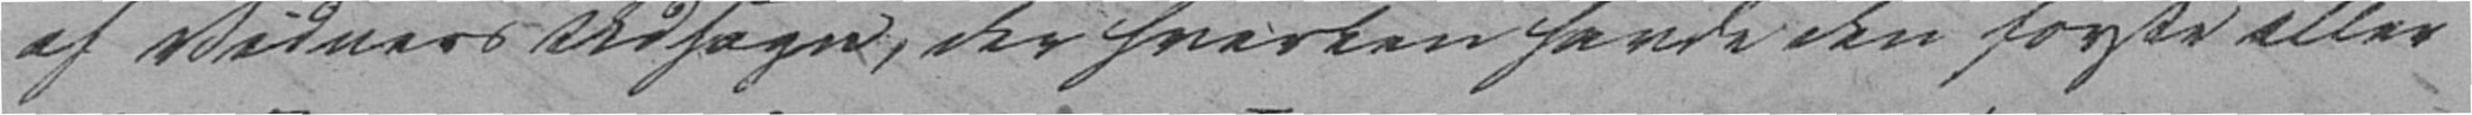af Vidners Udsagn, der hverken havde den første eller
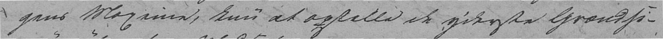gens Maxime, kun at opstille de yderste Grændse-
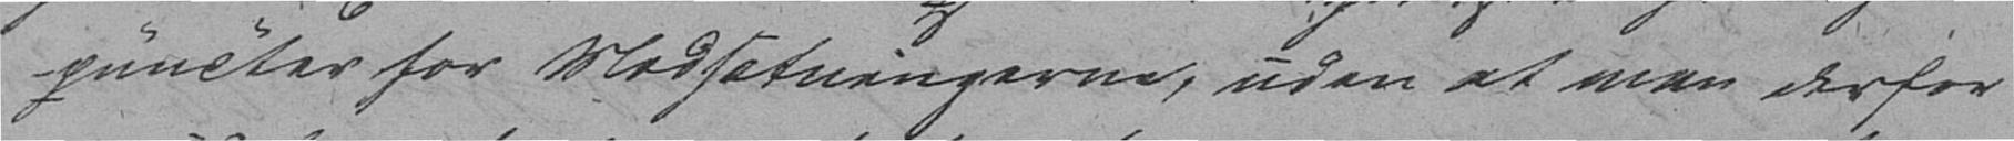puncter for Modsætningerne, uden at man derfor
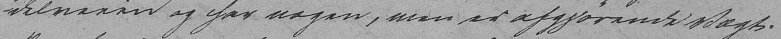delveien og har nogen, men ei afgjørende Vægt.
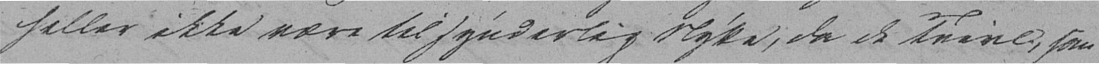heller ikke være til synderlig Nytte, da de Tvivl, som
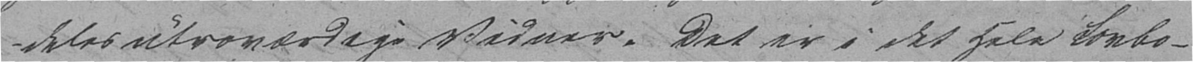deles utroværdige Vidner. Det er i det Hele Lovbo-
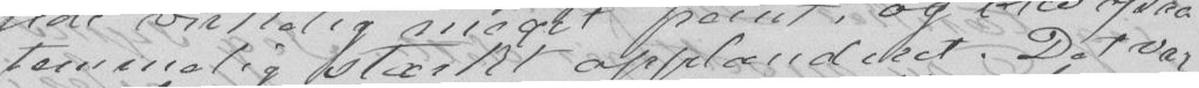temmelig stærkt applauderet. Det var
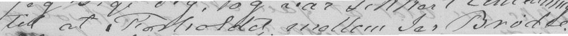til at Forholdet mellem Jer Brødre
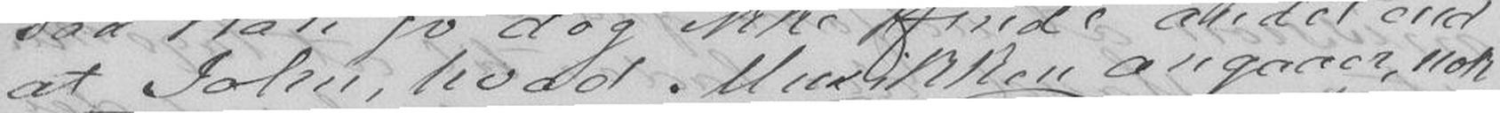at John, hvad Musikken angaaer, nok
